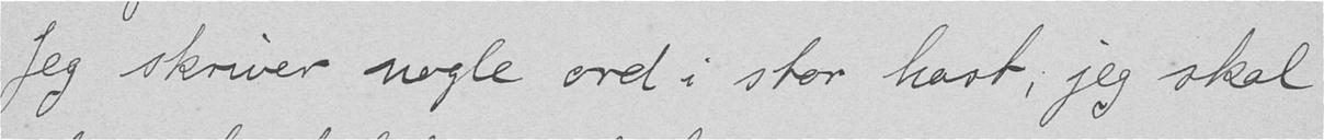Jeg skriver nogle ord i stor hast; jeg skal
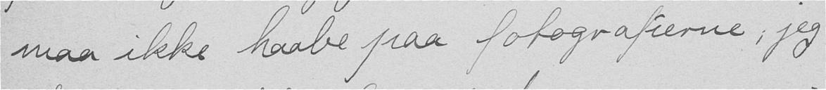maa ikke haabe paa fotografierne, jeg
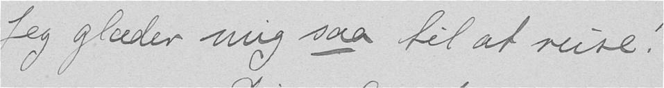Jeg glæder mig saa til at reise!
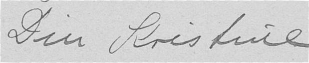Din Kristine
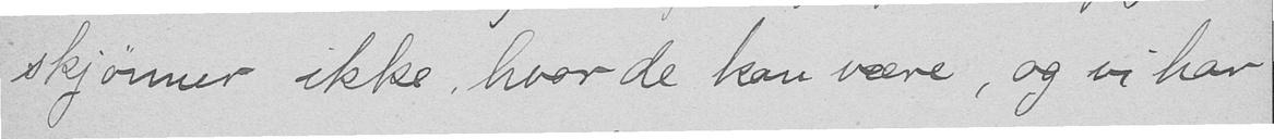skjønner ikke hvor de kan være, og vi har
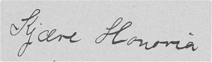Kjære Honoria
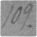109.
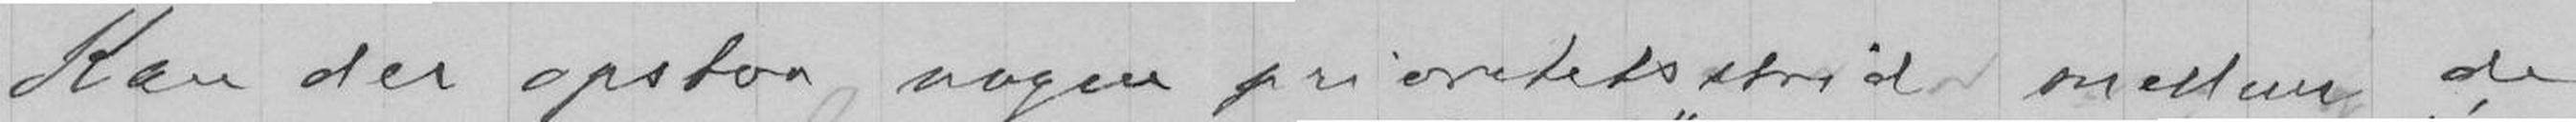Kan der opstaa nogen prioretetsstrid mellem de
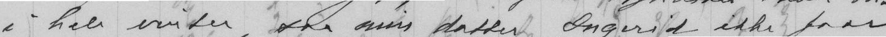i hele vinter, saa min datter Ingerid ikke faar
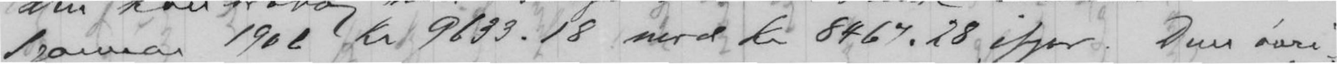1 januar 1906 kr 9633.18 mod kr 8467.28 ifjor. Deres øvri-
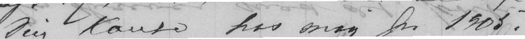Din Konto hos mig for 1905.
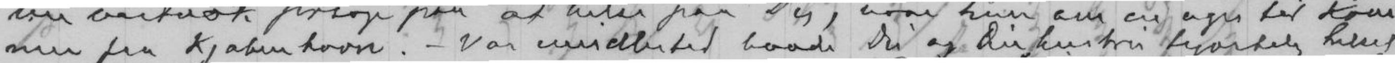mer fra Kjøbenhavn. - Vær imidlertid baade Du og Din hustru hjærtelig hilset
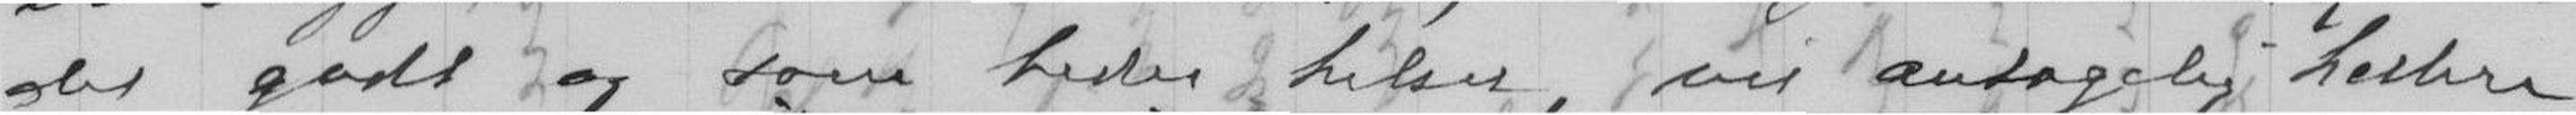det godt og som bedes hilses, vil antagelig hellere
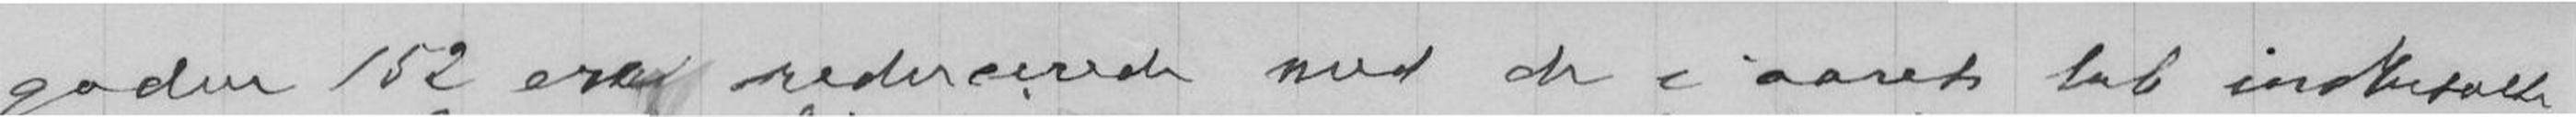gaden 152 ere reducerede med de i aarets løb indbetalte
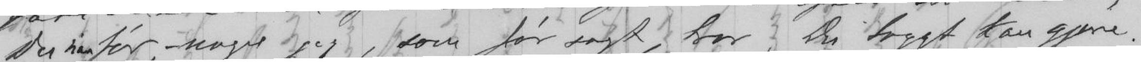Du har før, noget jeg, som før sagt, tror du trygt kan gjøre.
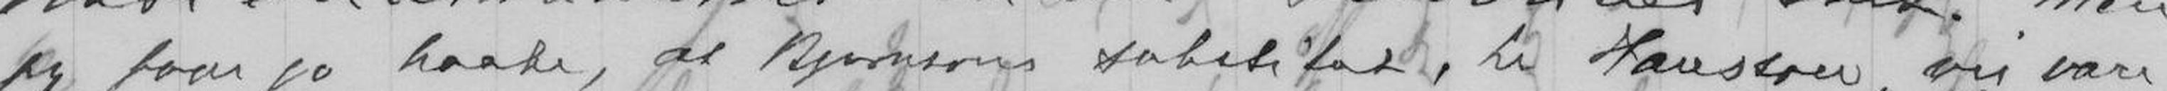jeg faar jo haabe, at Bjørnsons sobstifærd, til Hauston, vil være
